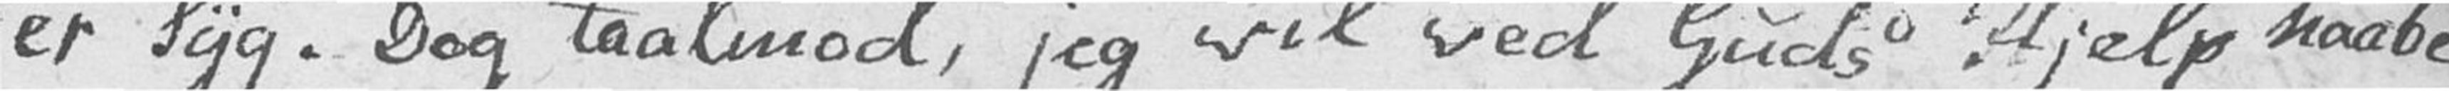er Syg. Dog taalmod, jeg vil ved Guds Hjelp haabe
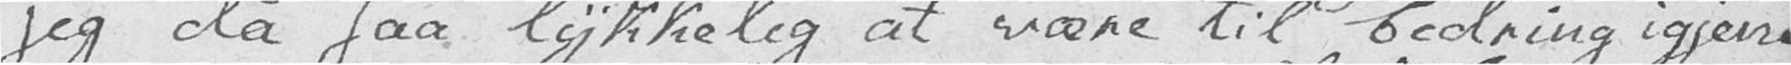jeg da saa lykkelig at være til bedring igjen.
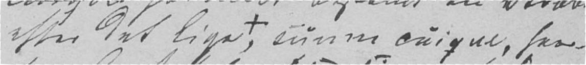efter det Lige, suum cuique, hvor-
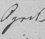Opret-
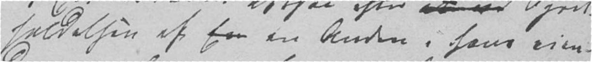holdelsen af Een en Anden i hans eien-
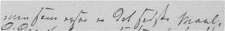men som ogsaa er det sidste Maal,
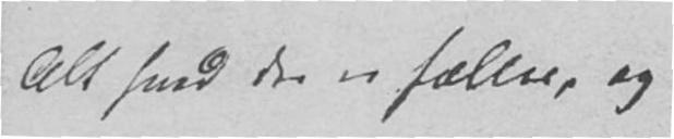Alt hvad der er fælles, og
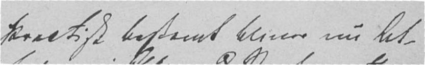Practisk bestemt bliver nu Ret-
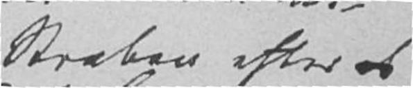Stræben efter et
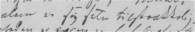alene er sig selv tilstrækkelig.
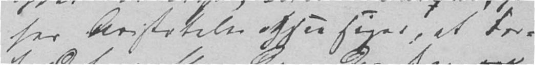for Aristoteles ogsaa siger, at For-
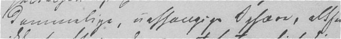dommelige, uafhængige Sphære, altsaa
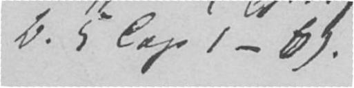B. 5 Cap 1-65).
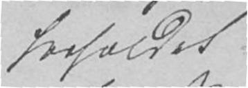forholdet
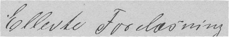Ellevte Forelæsning
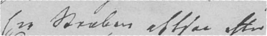En Stræben altsaa efter
